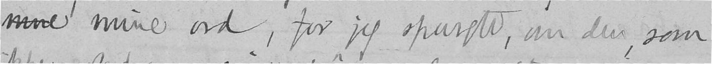mne mine ord, for jeg spurgte, om den, som
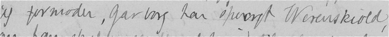jeg formoder, Garborg har spurgt Werenskiold,
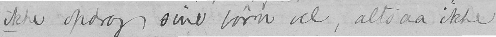ikke opdrog sine børn vel, altsaa ikke
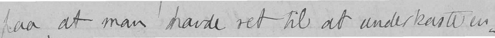paa, at man havde ret til at underkaste en-
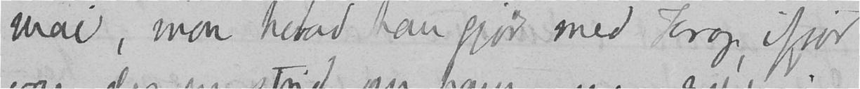mai, mon hvad han gjør med Krog, ifjor
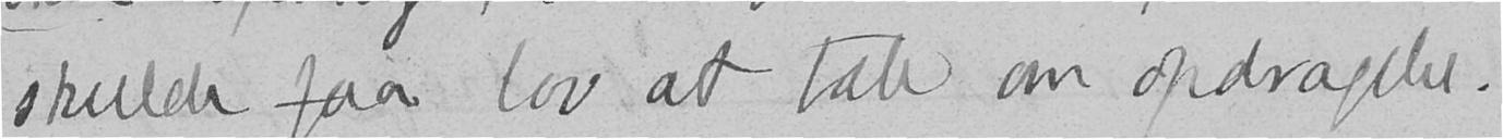skulde faa lov at tale om opdragelse.
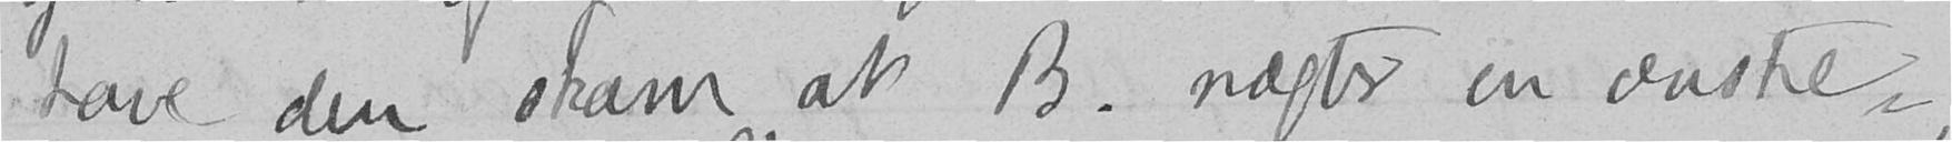have den skam, at B. nægter en venstre-
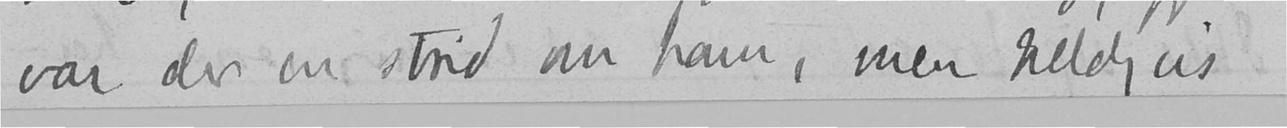var der en strid om ham, men heldigvis
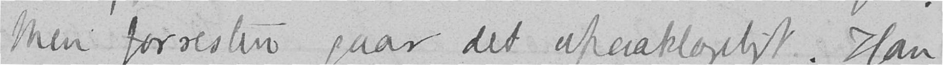Men forresten gaar det upaaklageligt. Han
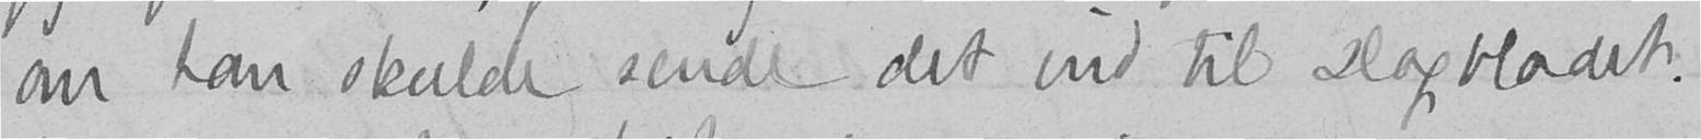om han skulde sende det ind til Dagbladet.
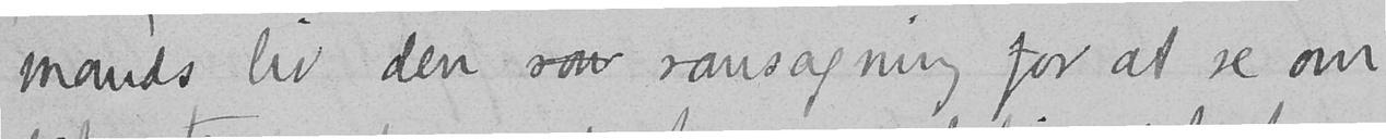mands liv den som ransagning for at se om
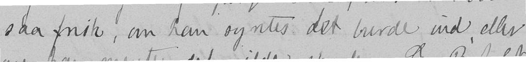saa frisk, om han syntes det burde ind, eller
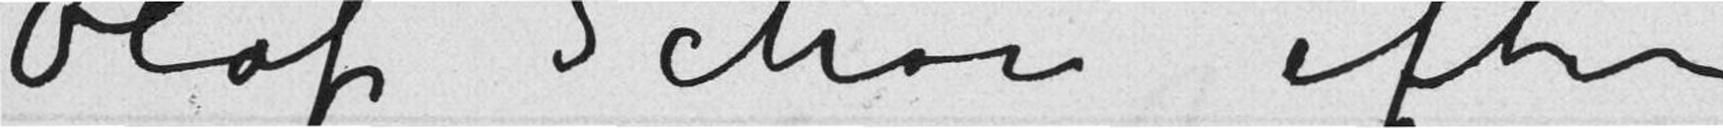Olaf Schou efter
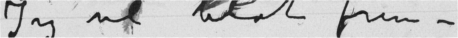Jeg vil blot frem-
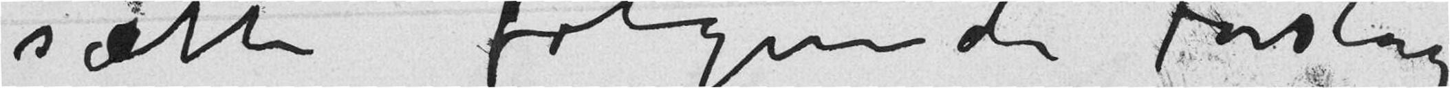sætte følgende Forslag
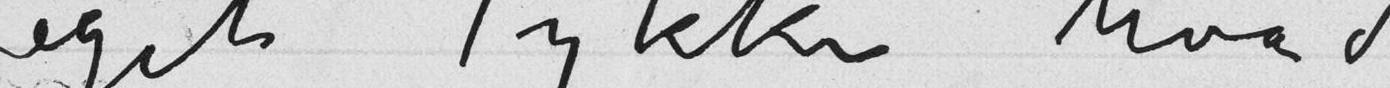eget Tykke hvad
